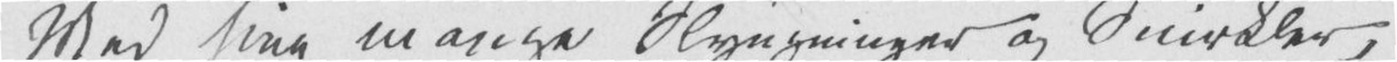Med hine mange Slyngninger og Snirkler,
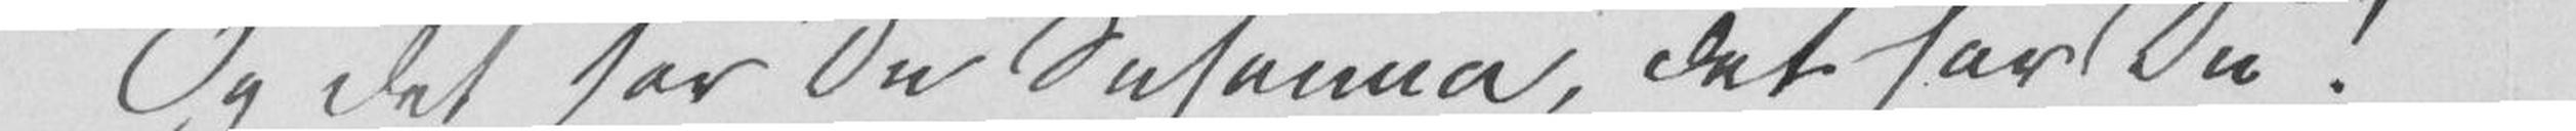Og det har Du Susanna, det har Du!
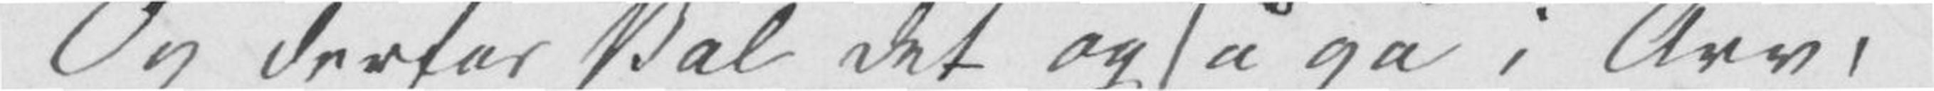Og derfor skal det også gå i Arv,
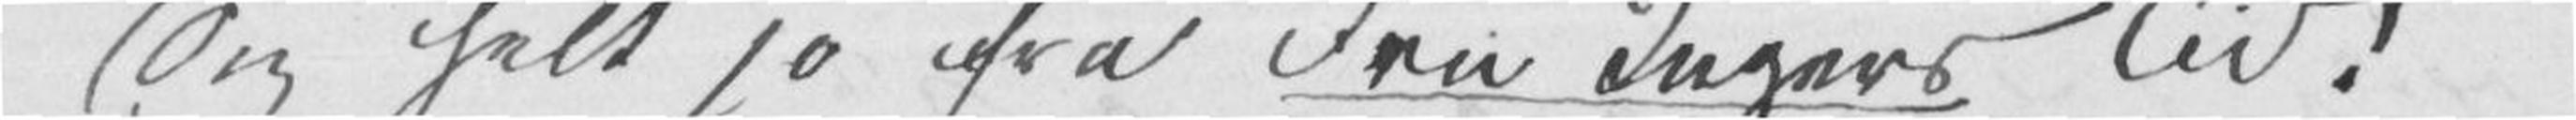Sig helt jo fra Fru Ingers Tid!
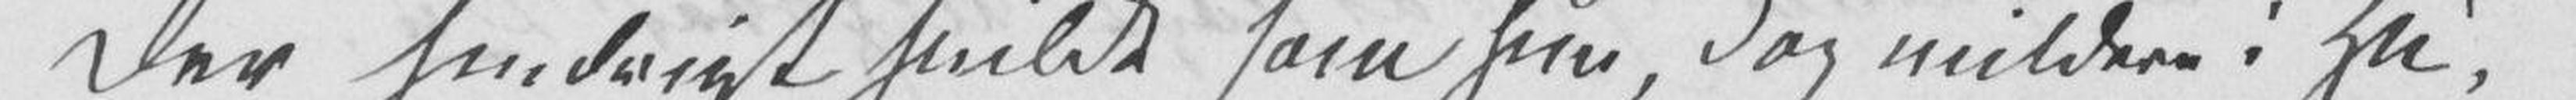Der sindrigt snildt som hun, dog mildere i Hu!
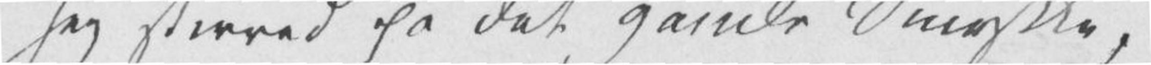Jeg stirred på det gamle Smykke,
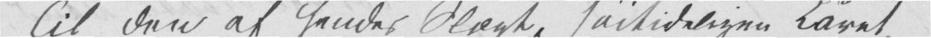Til den af hendes Slægt, høitideligen kåret,
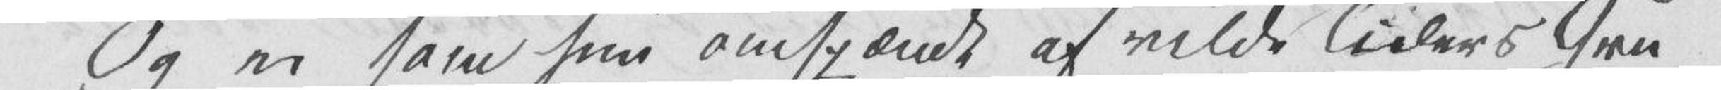Og vi som hun omspændt af vilde Tiders Gru,
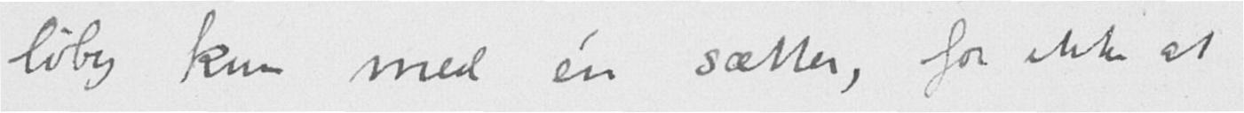løbig kun med én sætter, for ikke at
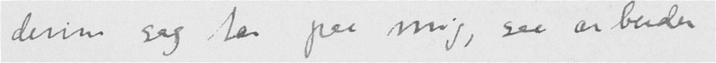denne sag tar paa mig, saa arbeider
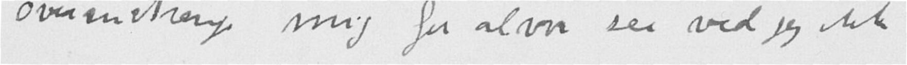overanstrænge mig for alvor saa ved jeg ikke
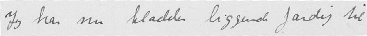Jeg har nu kladder liggende færdig til
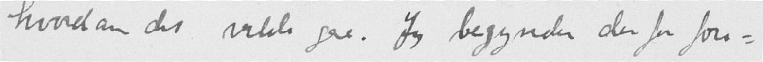hvordan det vilde gaa. Jeg begynder derfor fore-
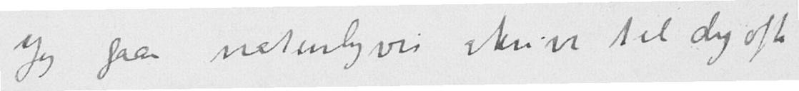Jeg faar naturligvis skrive til dig ofte
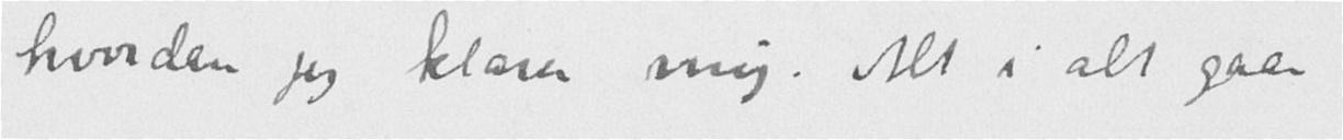hvordan jeg klarer mig. Alt i alt gaar
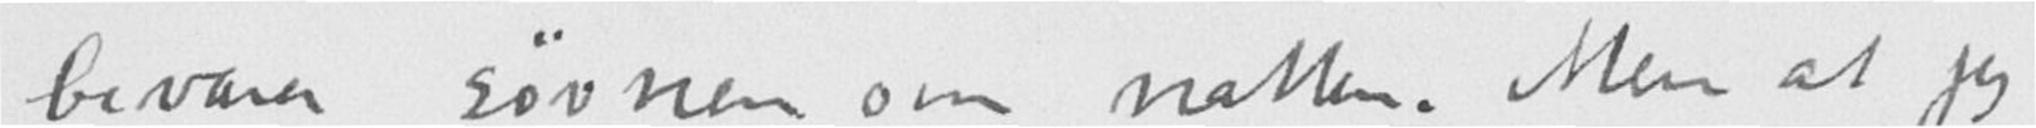bevarer søvnen om natten. Men at jeg
